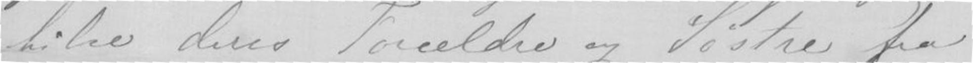hilse Deres For'ldre og Søstre fra
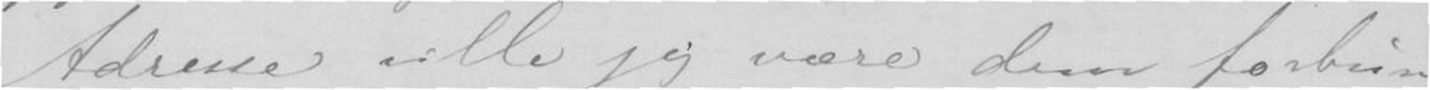Adresse ville jeg være dem forbun-
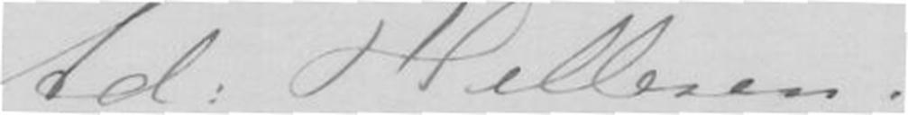Ad: J Hellesen.
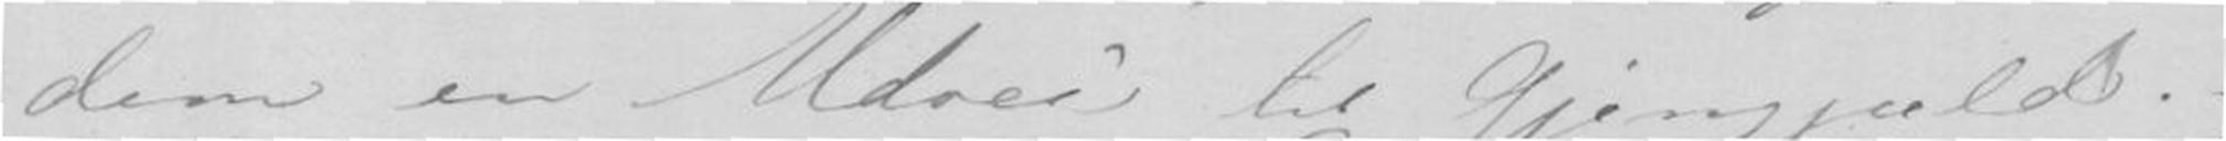dem en , til gjengjeld.-
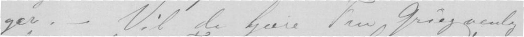ger.- Vil de kjære Fru Grieg venlig
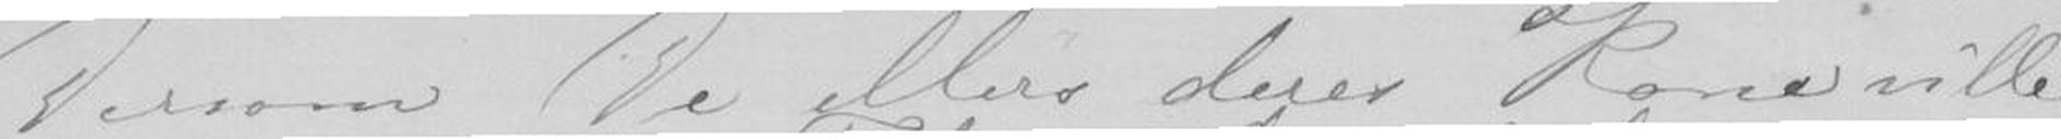Dersom De eller deres kone ville
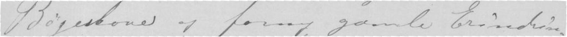Bøgeskone og fornye gamle Erindrin-
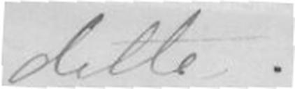dette.-
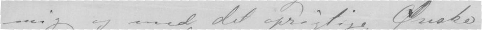mig og med det oprigtide Ønske
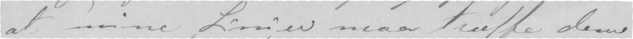at mine Linier maa træffe dem
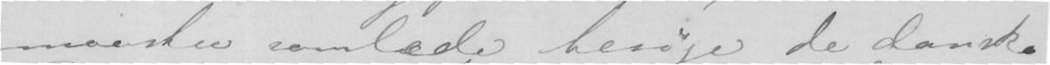næsten samlede besøge de danske
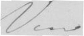Ven.
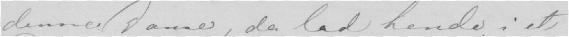denne Dame, da lad hende i et
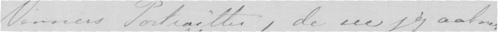Venners Portraitter, de ser jeg
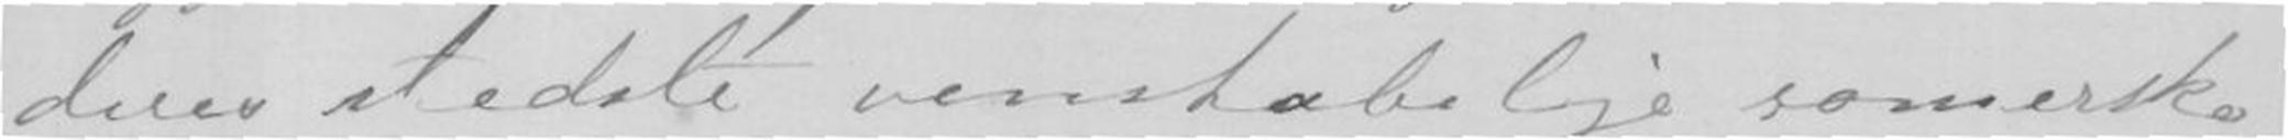deres venskabelige
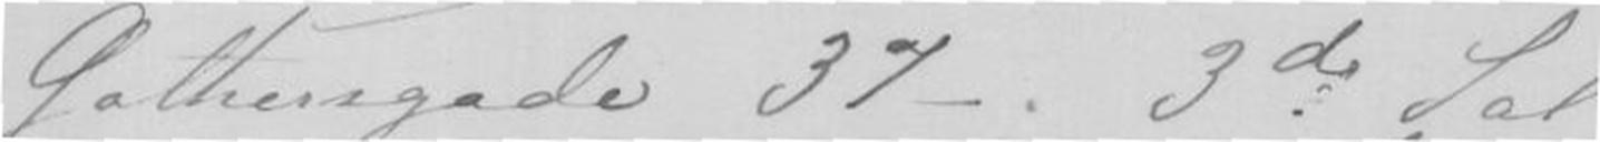Gathersgade 37-. 3de Sal
